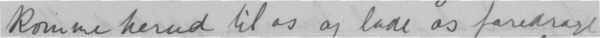komme herud til os og lade os foredrage
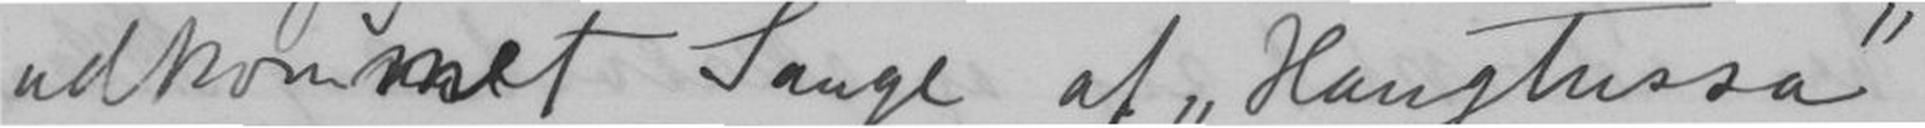udkommet Sange af "Haugtussa"
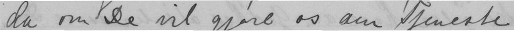da om De vil gjøre os den Tjeneste
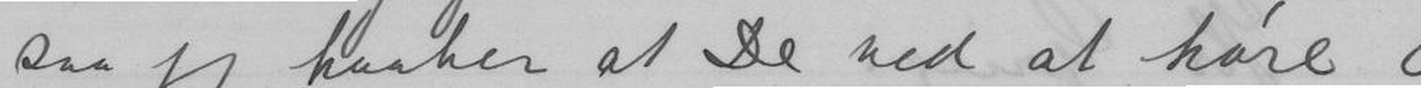saa jeg haaber at De ved at høre
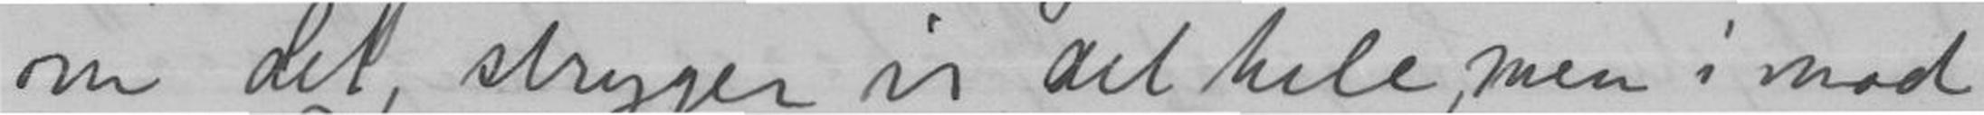om det, stryger vi det hele, men i mod-
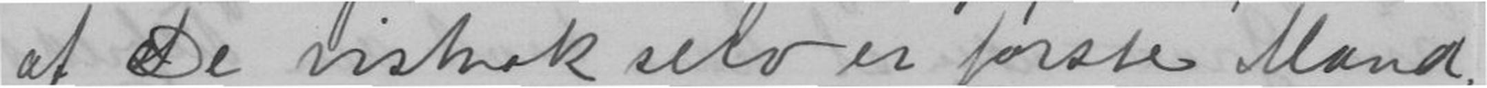af De vistnok selv er første Mand.
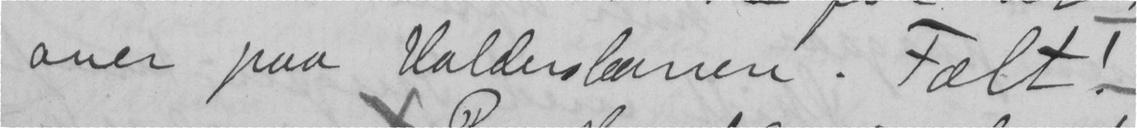over paa Valdersbanen. Fælt!
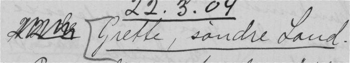Grette, søndre Land.
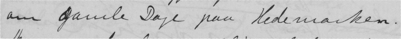om gamle Dage paa Hedemarken.
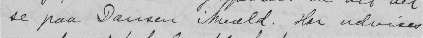se paa Dansen ikvæld. Her udvises
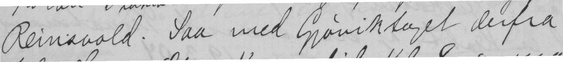Reinsvold. Saa med Gjøviktoget derfra
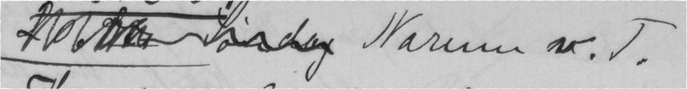Narum v. T.
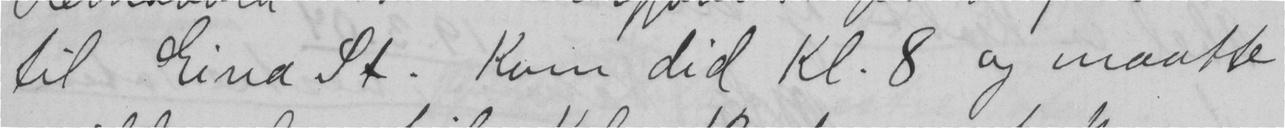til Eina St. Kom did Kl. 8 og maatte
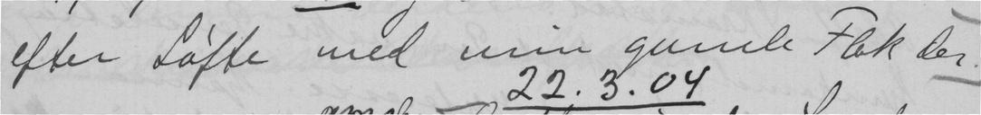efter Løfte med min gamle Flok der.
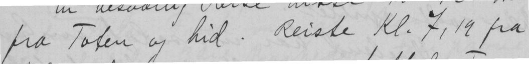fra Toten og hid. Reiste Kl. 7, 19 fra
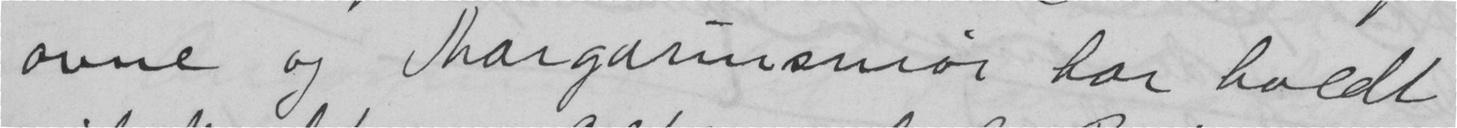ovne og Margarinsmør har holdt
